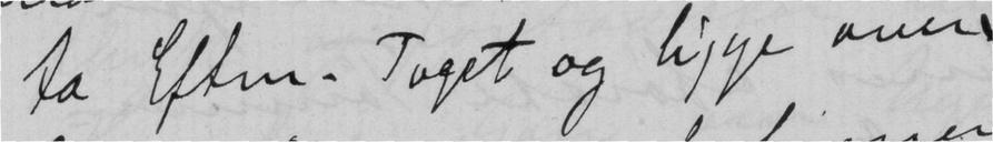ta Eftm. Toget og ligge over
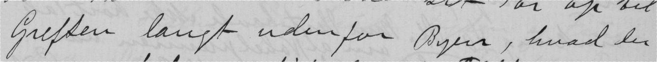Grefsen langt udenfor Byen, hvad der
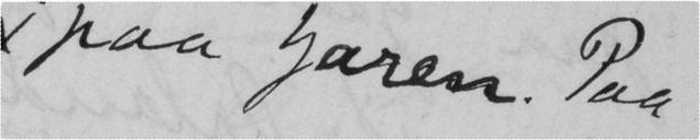paa Jaren. Paa
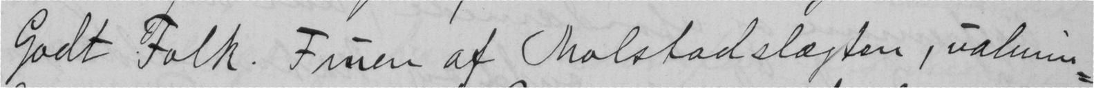Godt Folk. Fruen af Molstadslægten, ualmin-
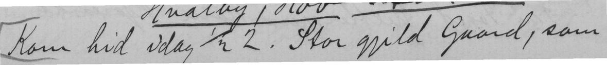Kom hid idag ½ 2. stor gjild Gaard, som
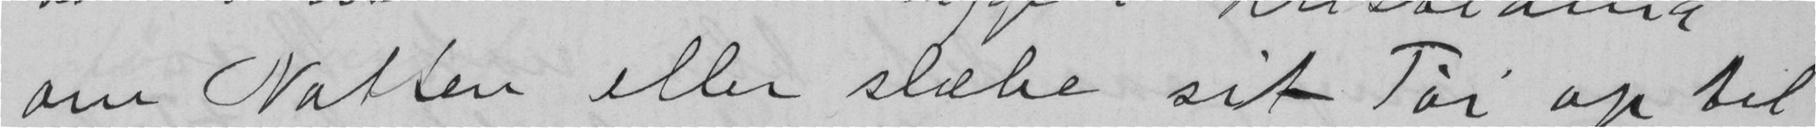om Natten eller slæbe sit Tøi op til
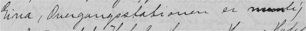Eina, Overgangsstationen er lig
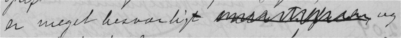er meget besværligt  og
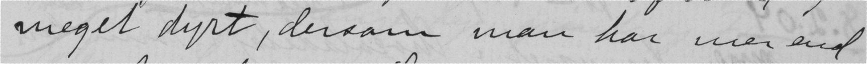meget dyrt, dersom man har mer end
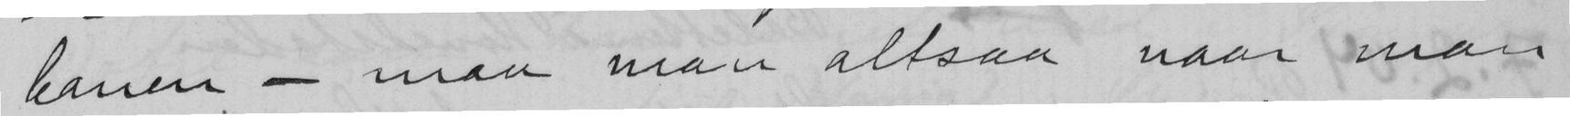banen - maa man altsaa naar man
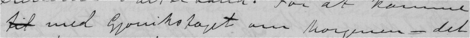til med Gjøvikstoget om Morgenen - det
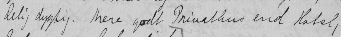delig dygtig. Mere godt Privathus end Hotel,
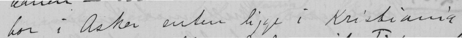bor i Asker enten ligge i Kristiania
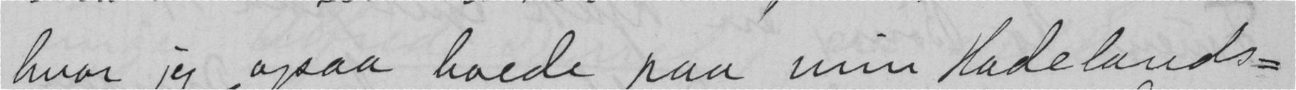hvor jeg ogsaa boede paa min Hadelands-
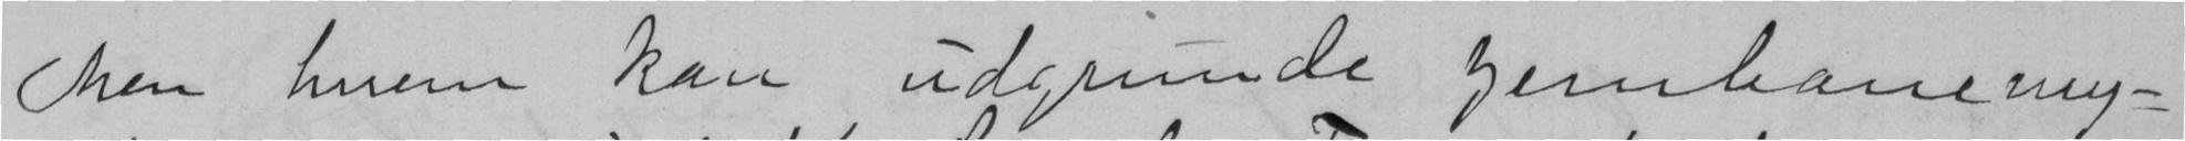Men hvem kan udgrunde Jernbane my-
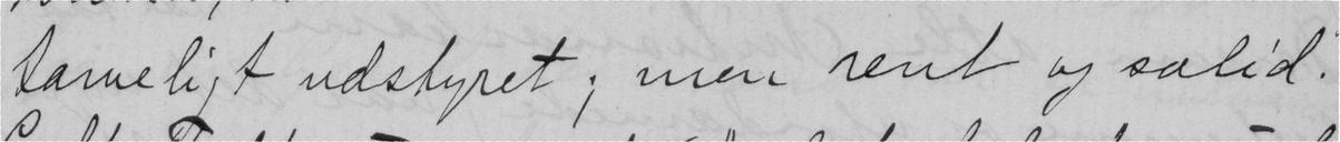tarveligt udstyret; men rent og solid.
